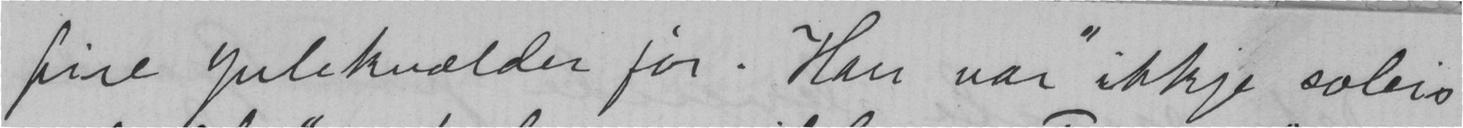fire julekvælder før. Han var "ikkje soleis
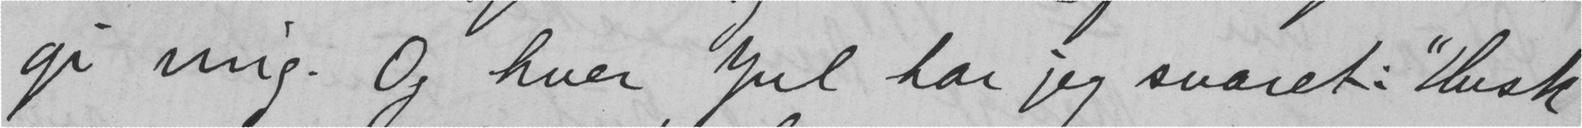gi mig. Og hver jul har jeg svaret: "Husk
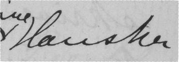Hansker
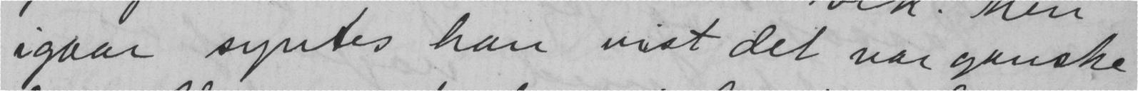igaar syntes han vist det var ganske
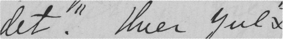det." Hver jul
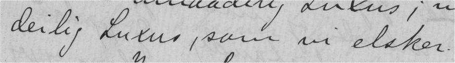deilig Luxus, som vi elsker.
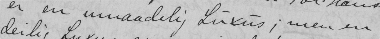er en umaadelig Luxus; men en
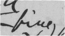fine
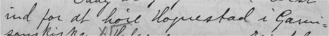ind for at høre Hognestad i Garni-
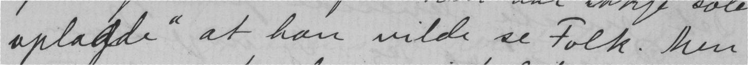oplagde" at han vilde se Folk. Men
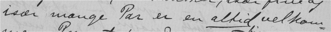især mange Par er en altid velkom-
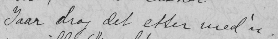I aar drog det etter med'en.
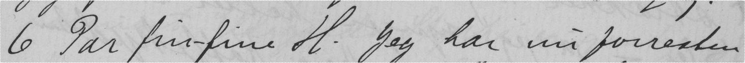6 Par fin-fine H. Jeg har nu forresten
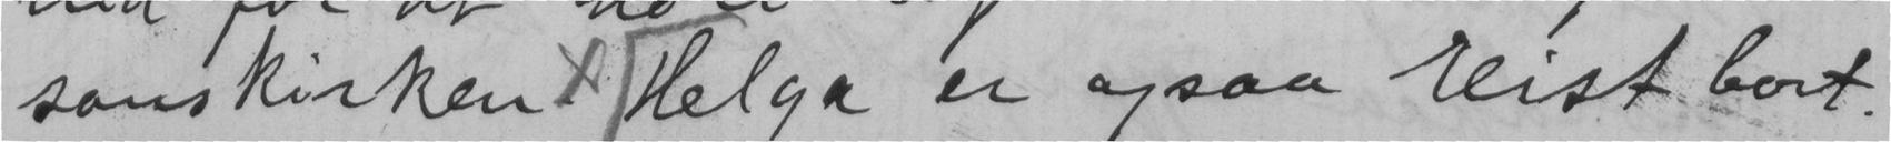sonskirken.x Helga er ogsaa reist bort.
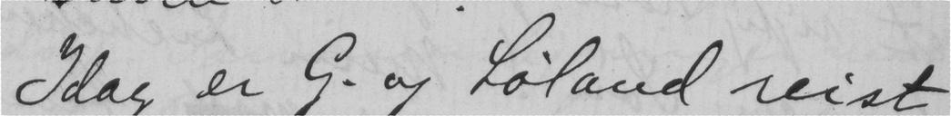Idag er G. og Løland reist
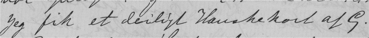Jeg fik et deiligt Hanskekort af G.
